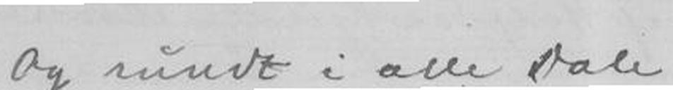Og rundt i alle Dale
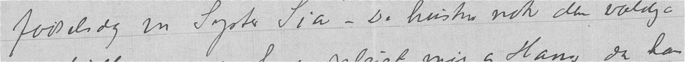fødselsdag var Syster Sia – De husker nok den vældige
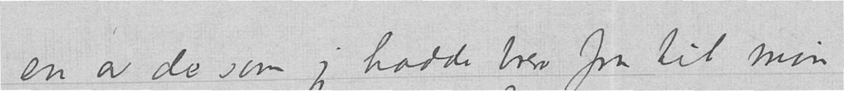en av de som jeg hadde brev fra til min
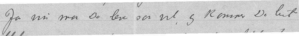Ja nu maa De leve saa vel, og kommer De hit
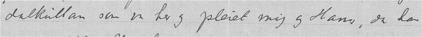dalkullan som var her og pleiet mig og Hans, da han
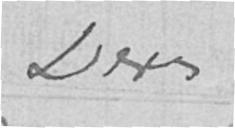Deres
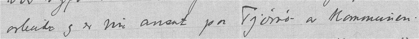arbeide og er nu ansat paa Tjømø av kommunen.
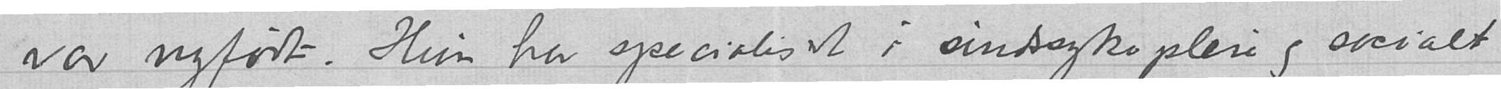var nyfødt. Hun har specialisert i sindssykepleie og socialt
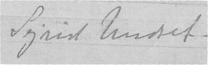Sigrid Undset
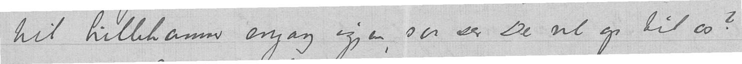til Lillehammer engang igjen, saa ser De vel op til os?
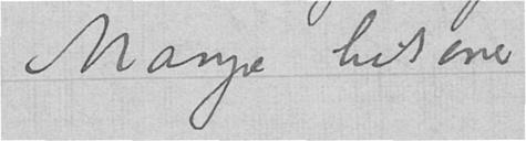Mange hilsener
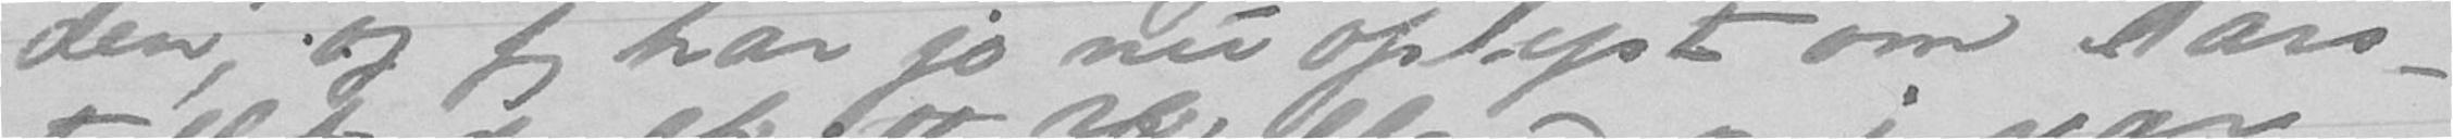den", og jeg har jo nu oplyst om Aars-
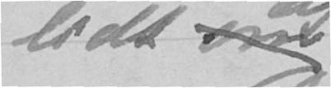lidt om
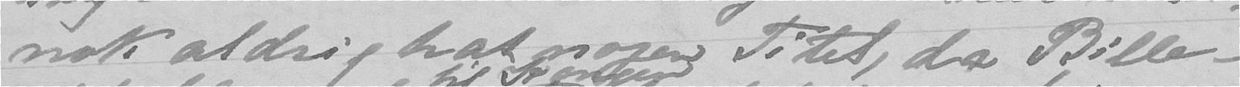nok aldrig hat nogen Titel, da Bille-
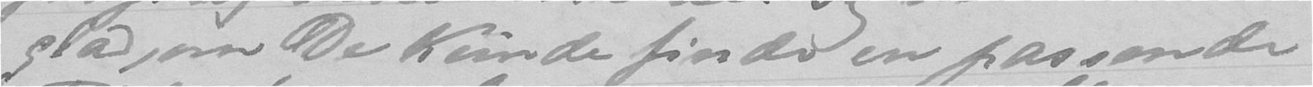glad, om De kunde finde en passende
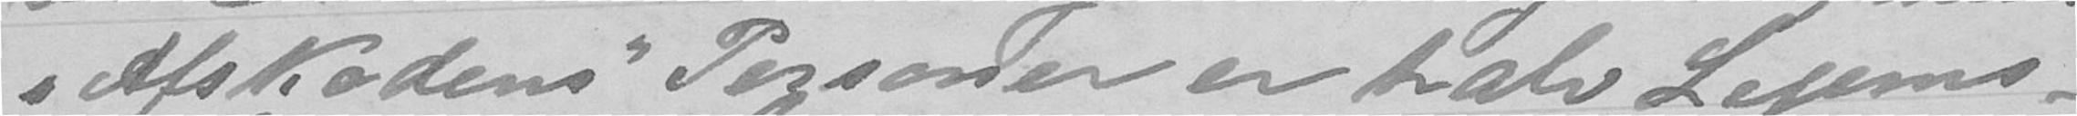"Afskedens" Personer er halv Legems-
